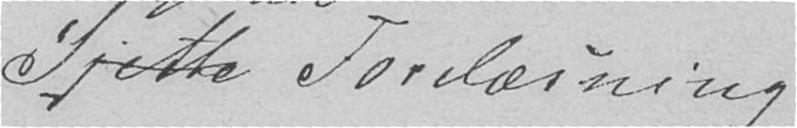Syvende Forelæsning
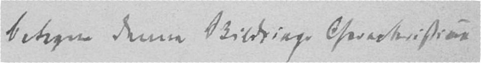betegne denne Skildrings Characteristica
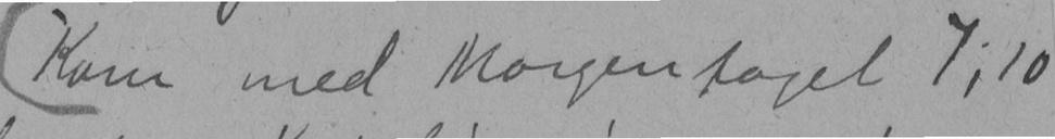Kom med Morgentoget 7:10
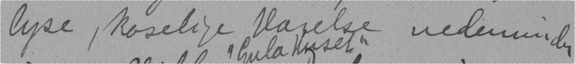lyse, koselige Værelse nedenunder
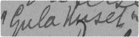"GulaHuset"
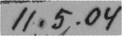11.5.04
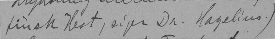finsk Hest, siger Dr. Hagelius.)
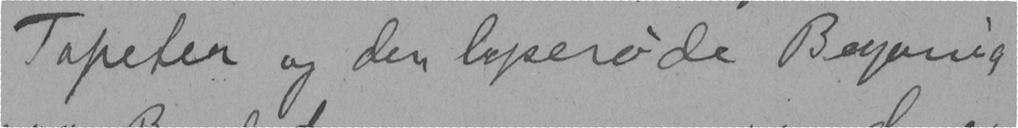Tapeter og den lyserøde Begonia
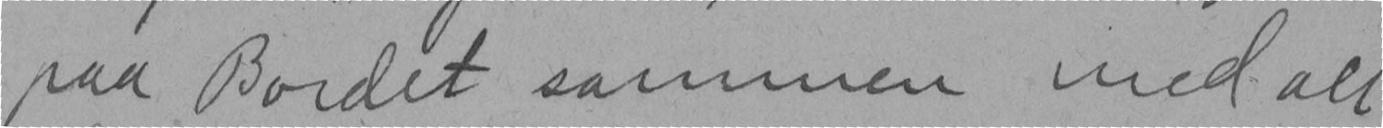paa Bordet sammen med alt
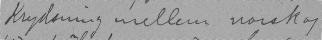Krydsning mellem norsk og
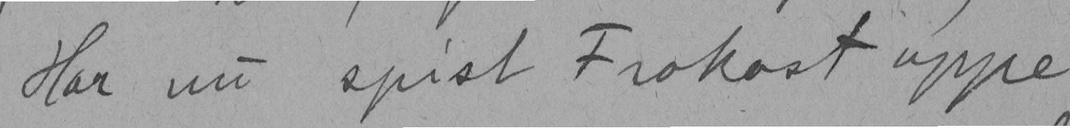Har nu spist Frokost oppe
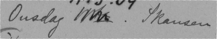Onsdag . Skansen
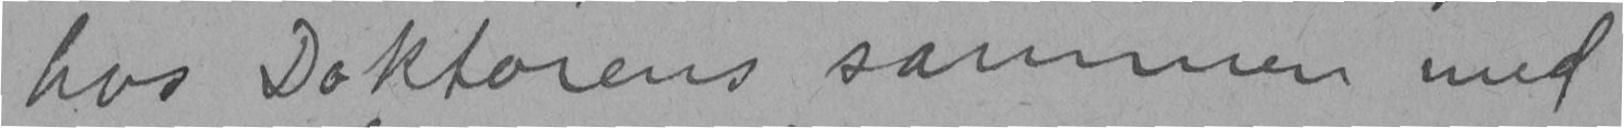hos Doktorens sammen med
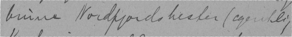brune Nordfjordshester (egentlig
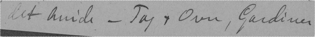det hvide - Tag, Ovn, Gardiner
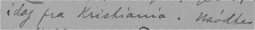idag fra Kristiania. Mødtes
